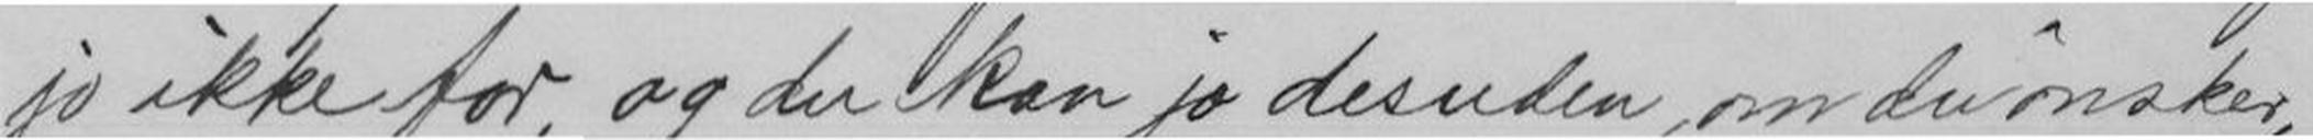jo ikke for, og du kan jo desuden, om du ønsker,
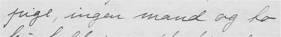pige, ingen mand og to
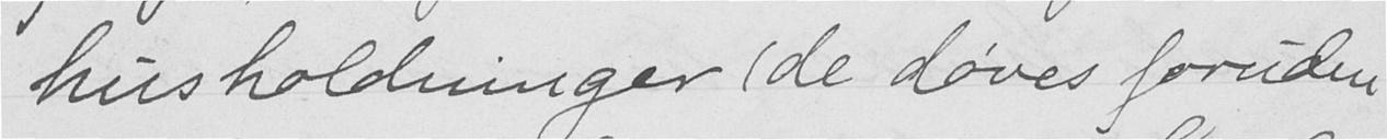husholdninger (de døves foruden
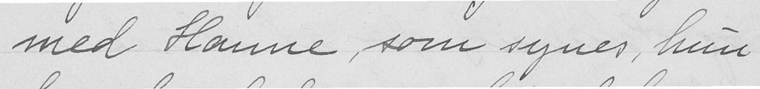Hanne, som synes, hun
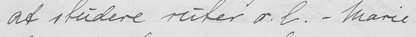at studere ruter o.l. - Marie
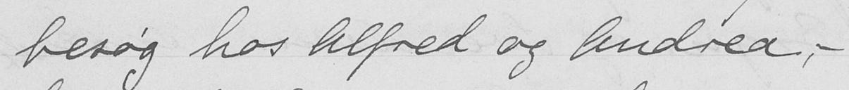besøg hos Alfred og Andrea, -
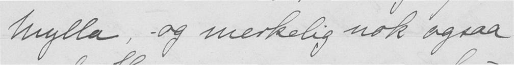hylla, og merkelig nok ogsaa
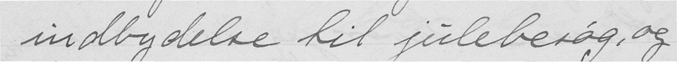indbydelse til julebesøg, og
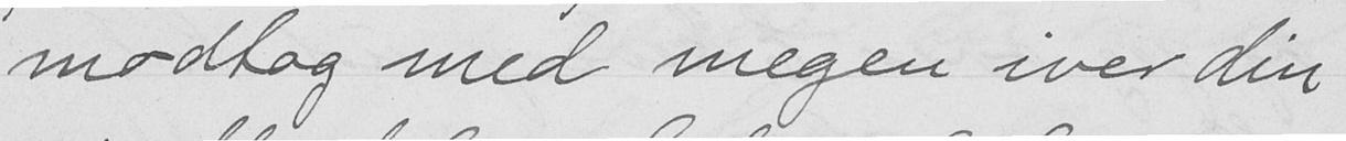modtog med megen iver din
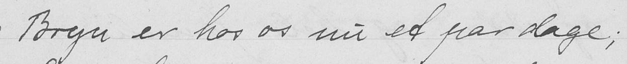Bryn er hos os nu et par dage;
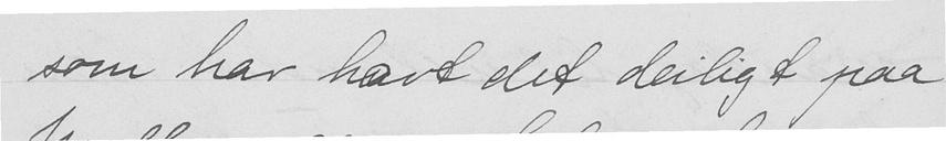som har havet det deiligt paa
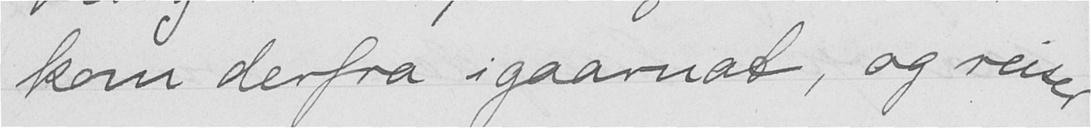kom derfra i gaarnat, og reiser
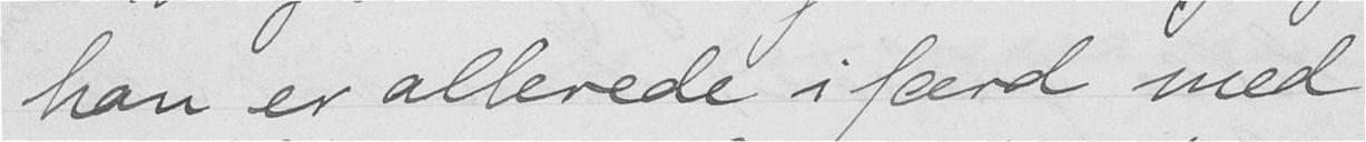han er allerede i færd med
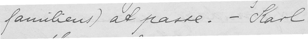familiens) at passe. - Karl
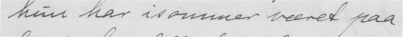hun har i sommer været paa
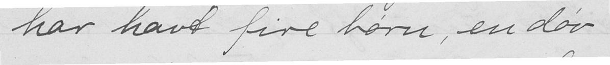har havet fire børn, en døv
